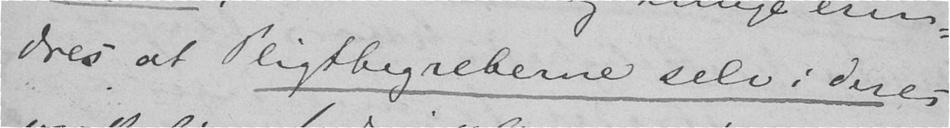dres at Pligtbegreberne selv i deres
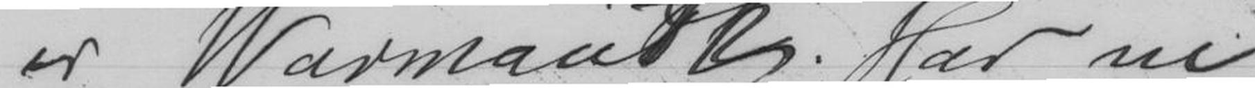er Warmauth. Har vi
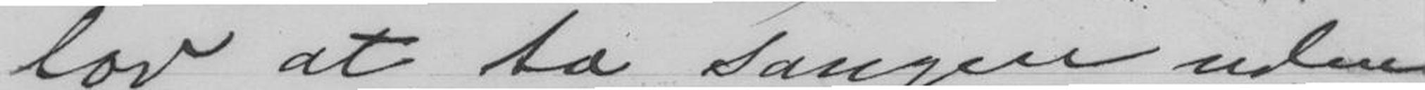lov at ta sangen uden
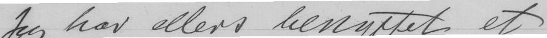Jeg har ellers benyttet et
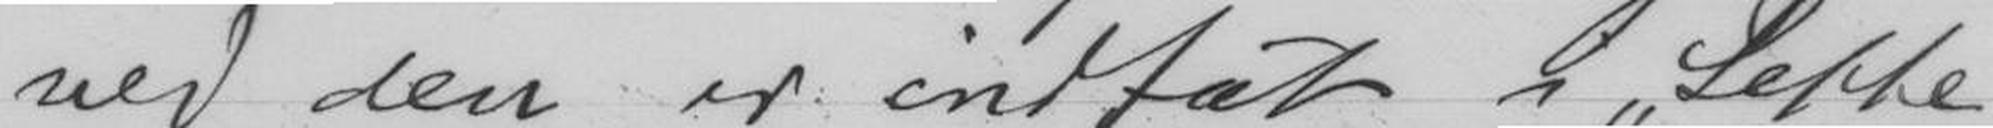ved den er indtat i Lette
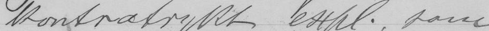kontratrykt expl., som
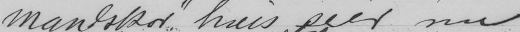Mandskor hvis sier nu
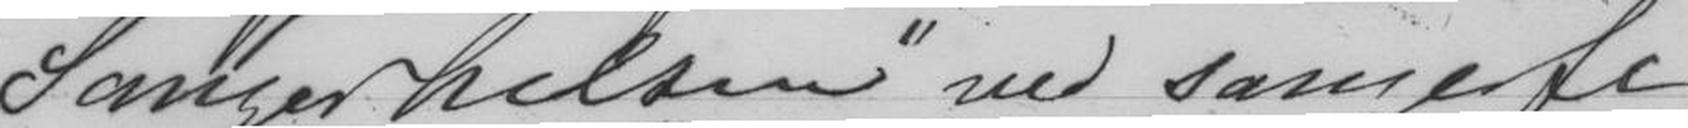Sangerhilsen ved sangerfe-
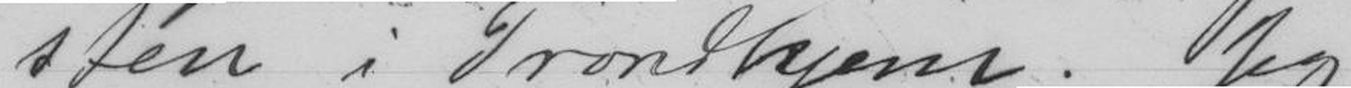sten i Trondhjem. Jeg
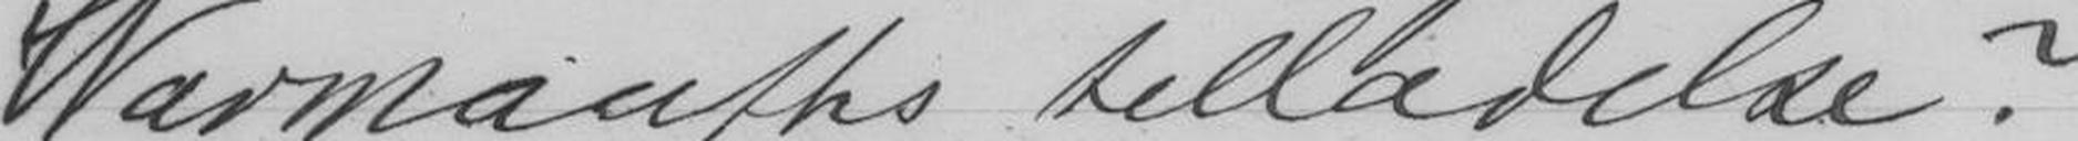Warmauths tilladelse?
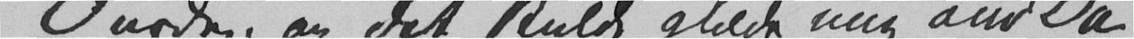Onsdag, og det skulde glæde mig om De
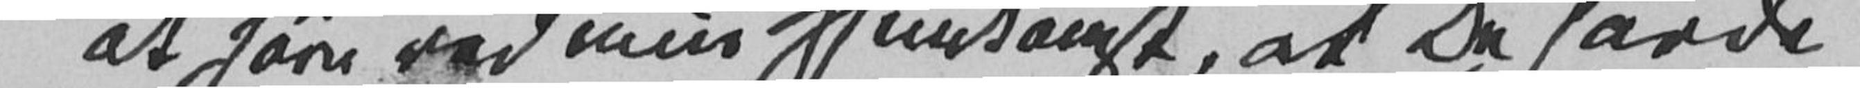at høre ved min Hjemkomst, at De havde
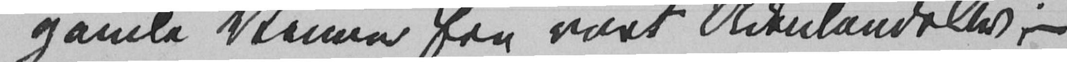gamle Venner fra vort Udenlandsliv –
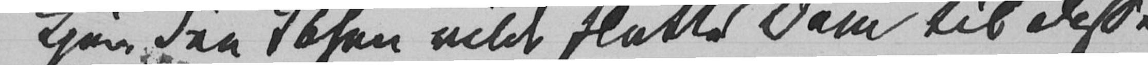kjere Fru Ibsen vilde sluttet Dem til disse
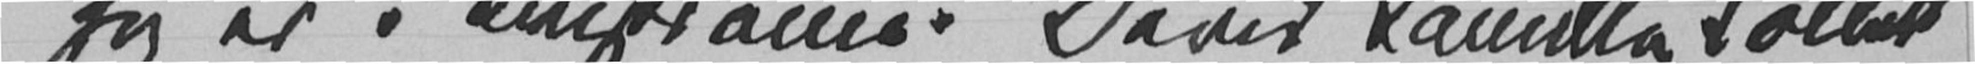«jeg er i Kristiania». Deres Camilla Collett
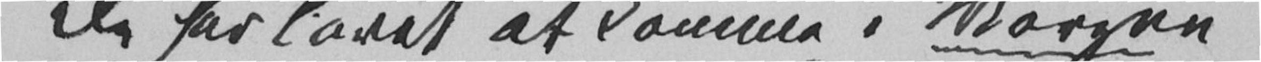De har lovet at komme i Morgen
